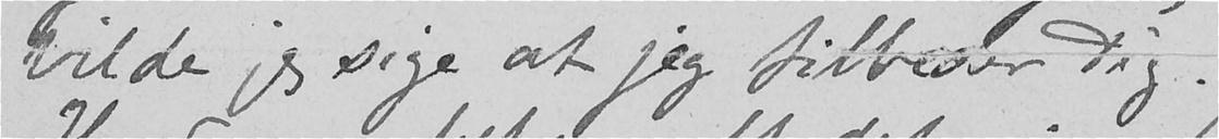vilde jeg sige at jeg tilbeder Dig.
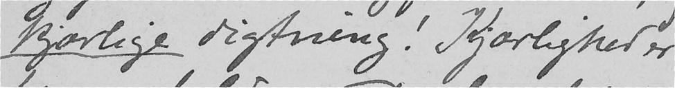kjærlige digtning! Kjærlighed er
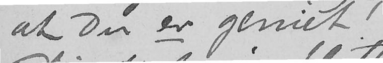at Du er geniet!
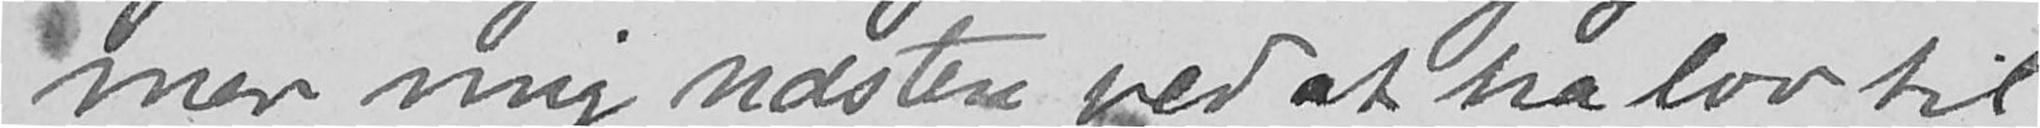mer mig næsten ved at ha lov til
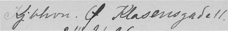Kjbhvn. Ø Klasensgade 11.
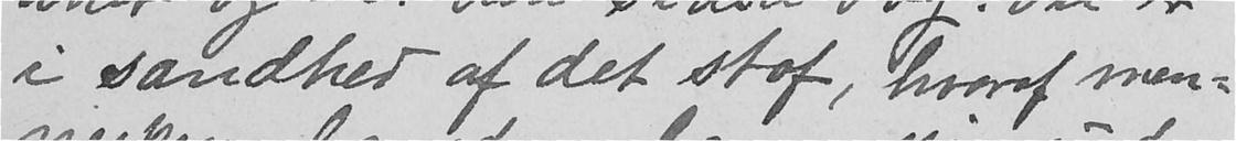i sandhed af det stof, hvoraf men-
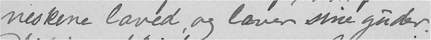neskene laved og laver sine guder.
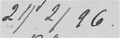21/2/96.
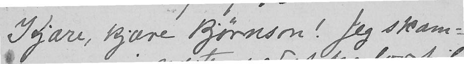Kjære, kjære Bjørnson! Jeg skam-
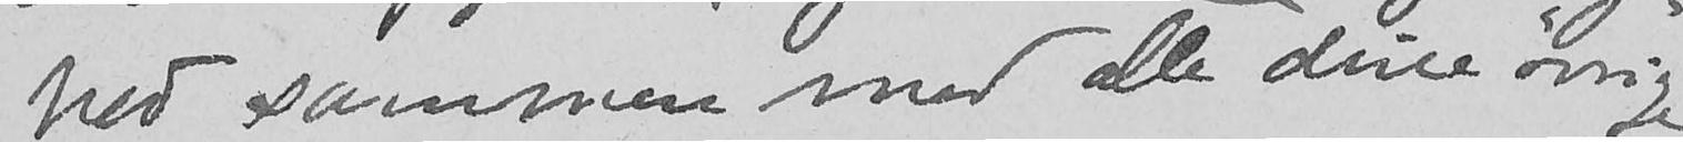hed sammen med alle dine øvrige
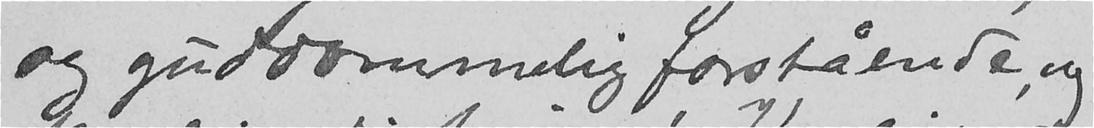og guddommelig forstående, og
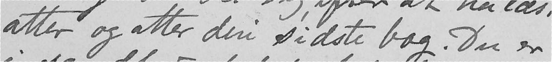atter og atter din sidste bog. Du er
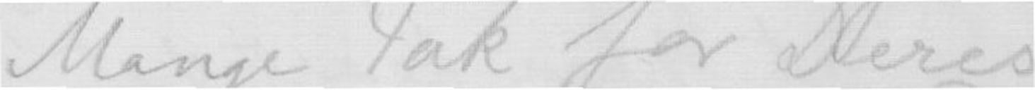Mange Tak for Deres
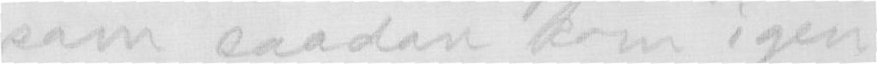som saadan kom igen.
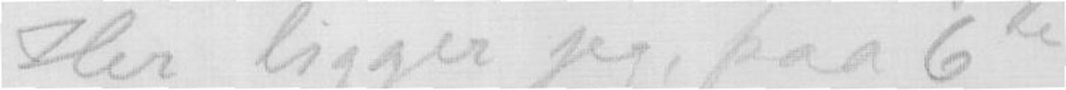Her ligger jeg, paa 6te
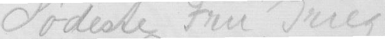Sødeste Fru Grieg!
